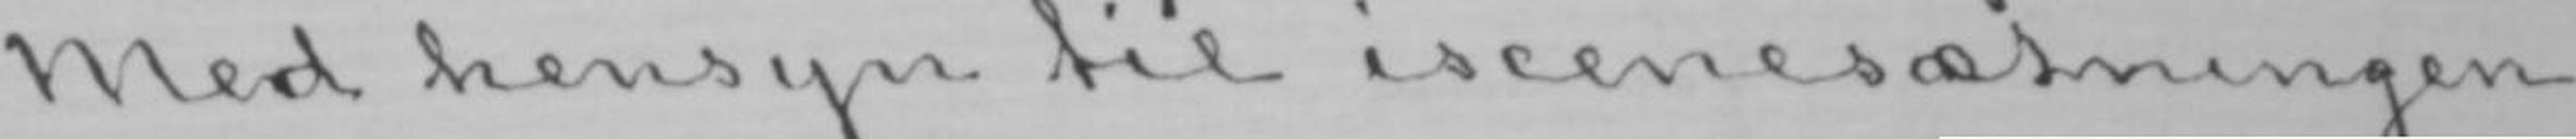Med hensyn til iscenesætningen
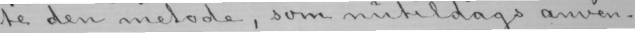te den metode, som nutildags anven-
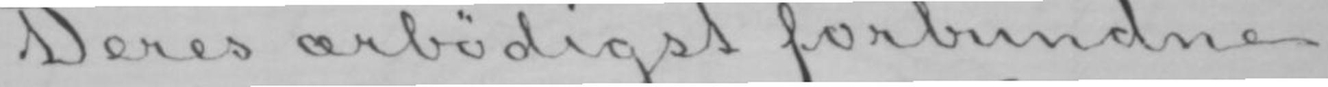Deres ærbødigst forbundne
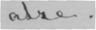atre.
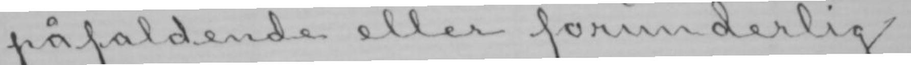påfaldende eller forunderlig.
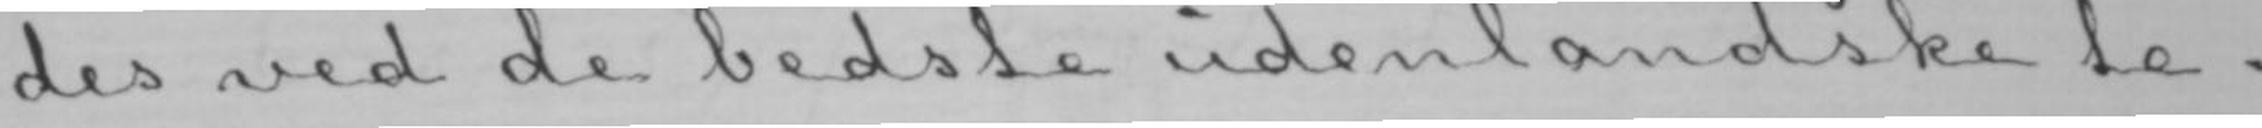des ved de bedste udenlandske te-
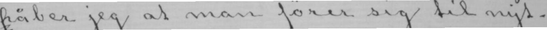håber jeg at man fører sig til nyt-
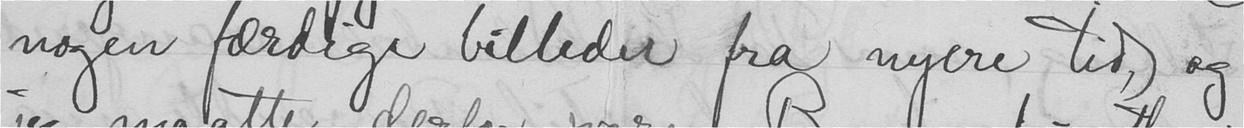nogen færdige billeder fra nyere tid, og
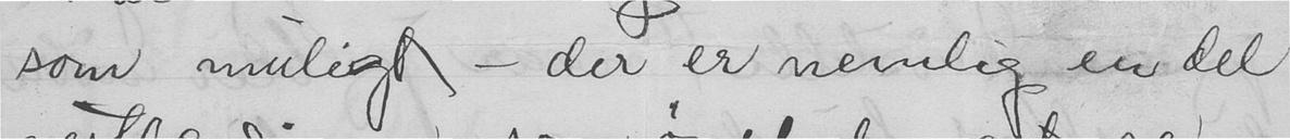som muligt - der er nemlig en del
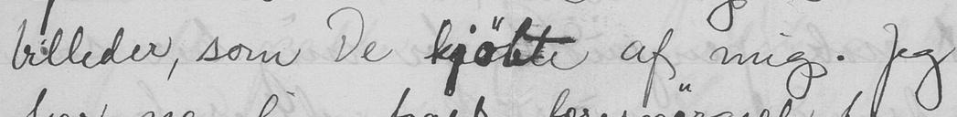billeder, som De kjøbte af mig. Jeg
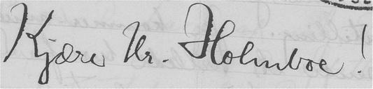Hjære Hr. Holmboe!
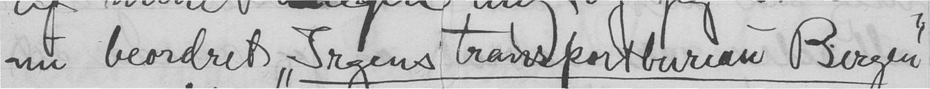nu beordret "Irgens transportbureau Bergen"
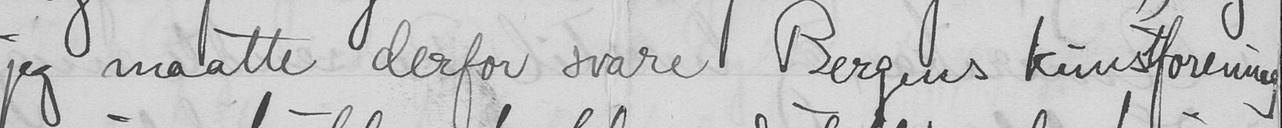jeg maatte derfor svare Bergens kunstforening
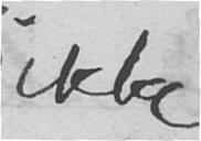ikke
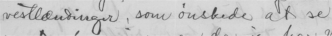vestlændinger, som ønskede at se
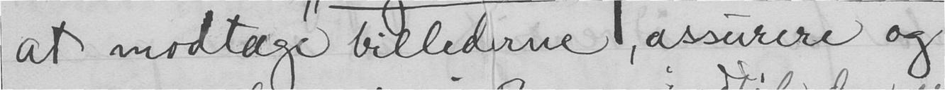at modtage billederne, assurere og
